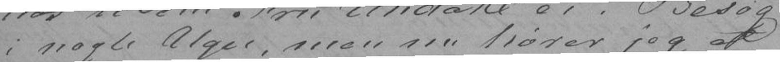i nogle Uger, men nu hører jeg at
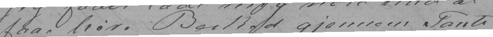faae høre Besked gjennem Tante
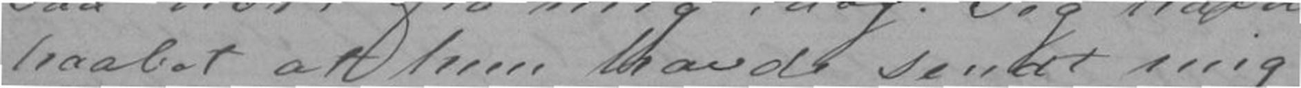haabet at hun havde sendt mig
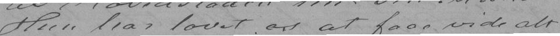Hun har lovet os at faae vide alt
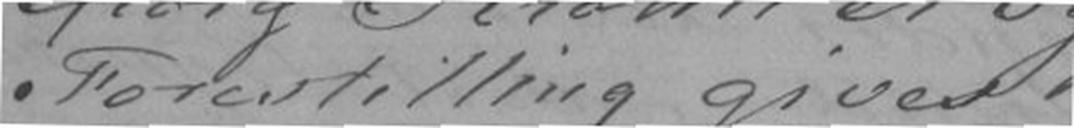Forestilling gives.
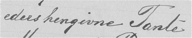eders hengivne Tante
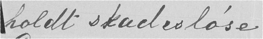holdt skadesløse.
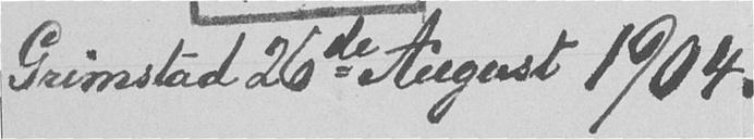Grimstad 26de August 1904.
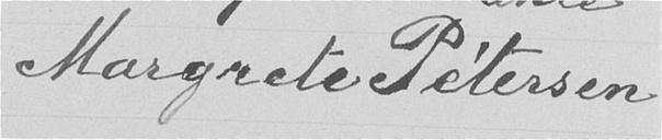Margrete Petersen
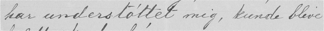har understøttet mig, kunde blive
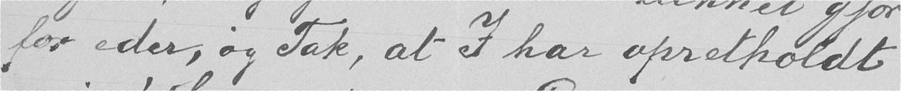for eder, og Tak, at I har opretholdt
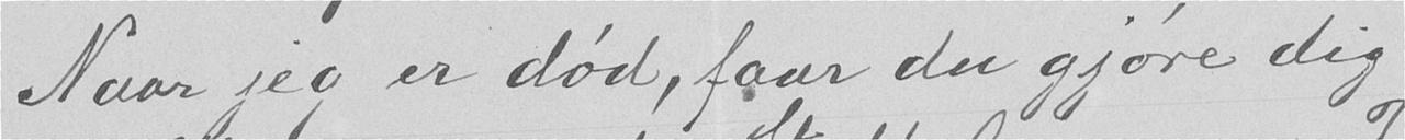Naar jeg er død, faar du gjøre dig
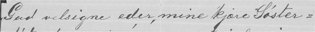Gud velsigne eder, mine kjære Søster-
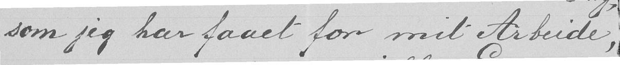som jeg har faaet for mit Arbeide,
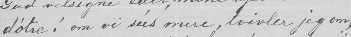døtre; om vi sees mere, tvivler jeg om,
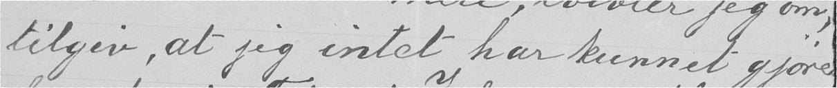tilgiv, at jeg intet har kunnet gjøre
